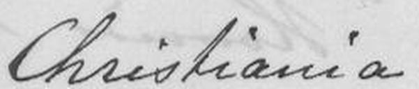Christiania
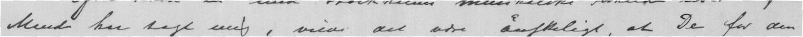Mend har sagt mig, vilde det være vanskeligt, at De for den
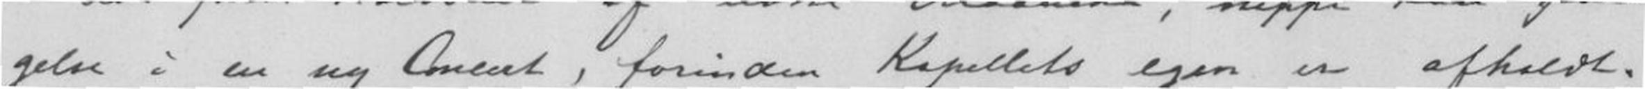gelse i en ny Consert, forinden Kapellets egen er afholdt.
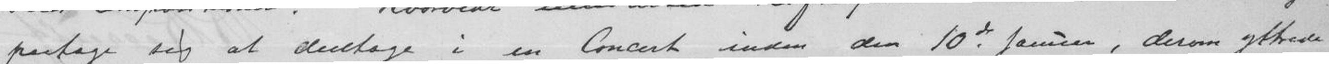paatage seg at tage i en Concert inden den 10de januar, dersom
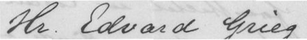Hr. Edvard Grieg
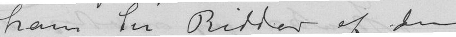ham til Ridder af den
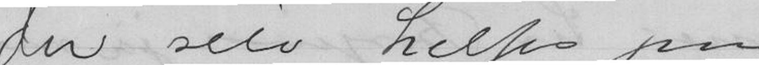du selv hilses paa
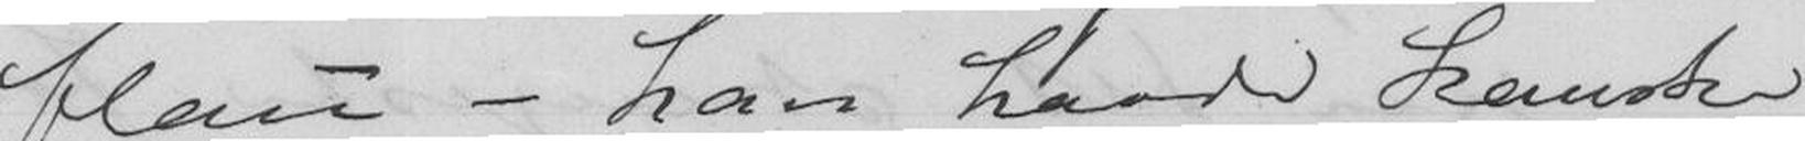flau - han havde kanske
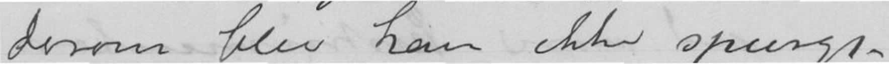derom blev han ikke spurgt.
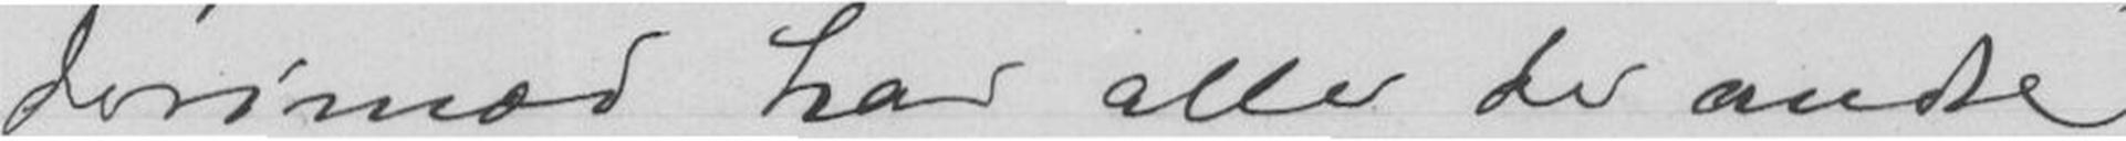derimod har alle de andre
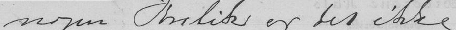nogen Kritik er det ikke
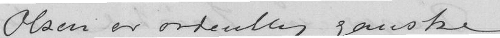Olsen er ordentlig ganske
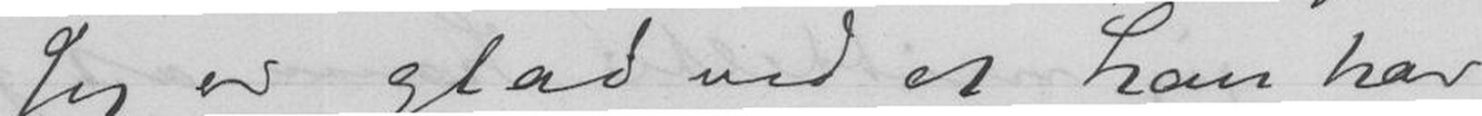Jeg er glad ved at han har
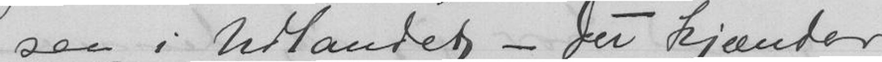saa i Udlandet - Du kjænder
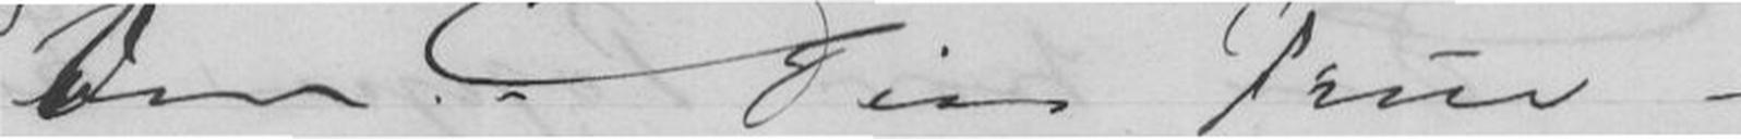Ven. Din Frue og
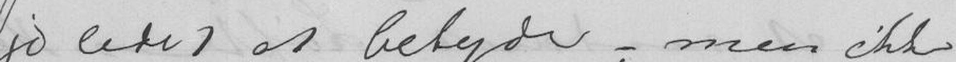jo lidet at betyde - men ikke
